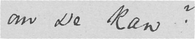om De kan?
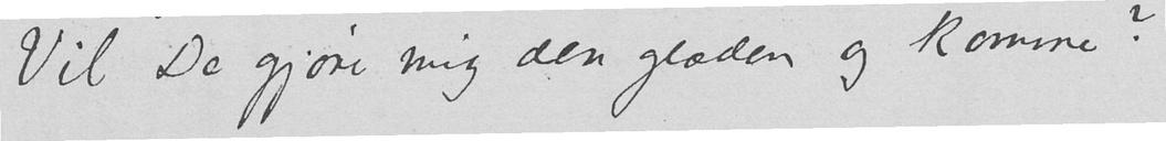Vil De gjøre mig den glæden og komme?
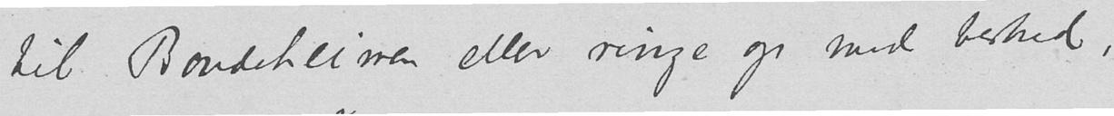til Bondeheimen eller ringe op med besked,
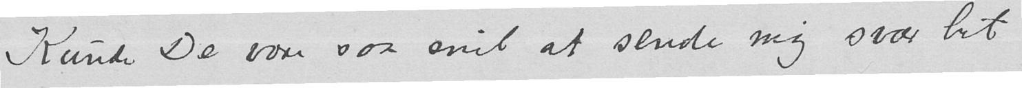Kunde De være saa snil at sende mig svar hit
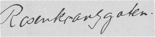Rosenkranzgaten.
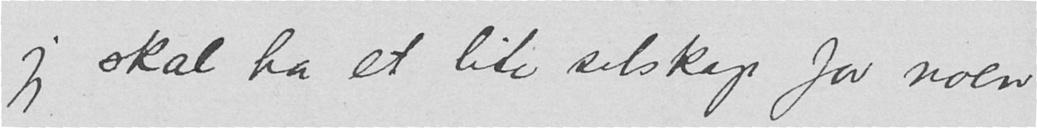jeg skal ha et lite selskap for noen
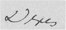Deres
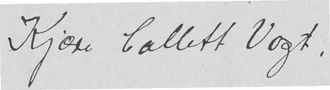Kjære Collett Vogt,
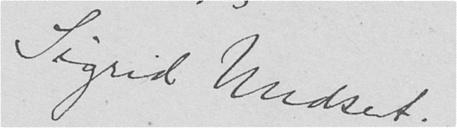Sigrid Undset.
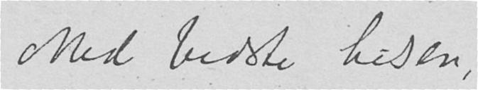Med bedste hilsen,
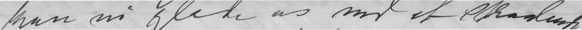kan vi glæde os med et straalende
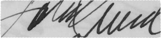John Lund
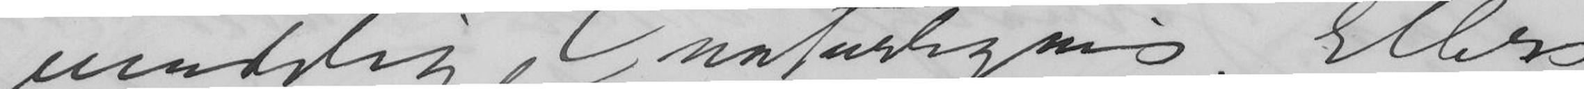uendelige naturligvis. Ellers
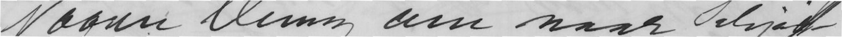Nogen Mening om naar Schjøtt
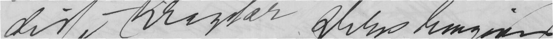disse Bragder Deres hengivne
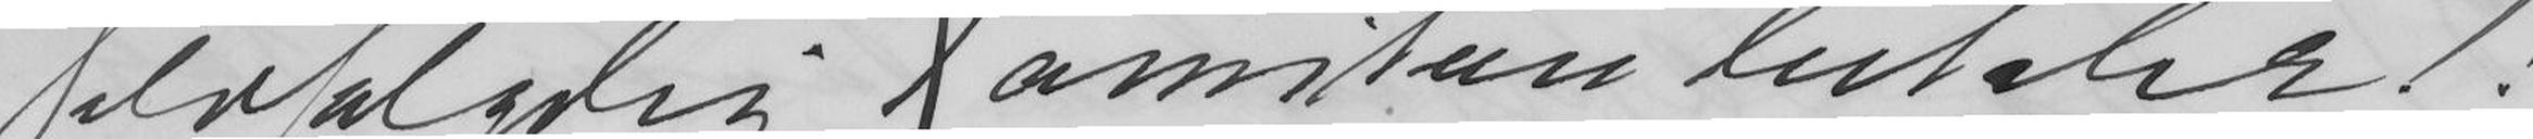selvfølgelig Komiteen betaler!!
